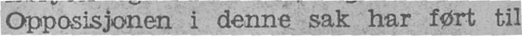Opposisjonen i denne sak har ført til
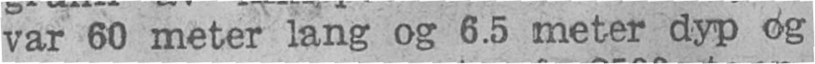var 60 meter lang og 6.5 meter dyp og
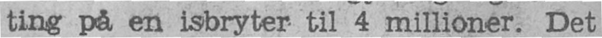ting på en isbryter til 4 millioner. Det
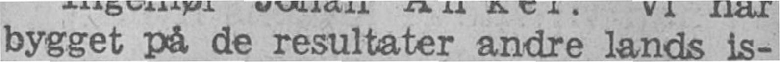bygget på de resultater andre lands is-
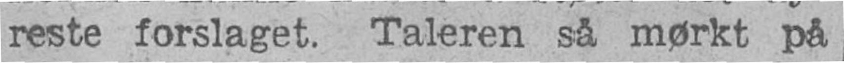reste forslaget. Taleren så mørkt på
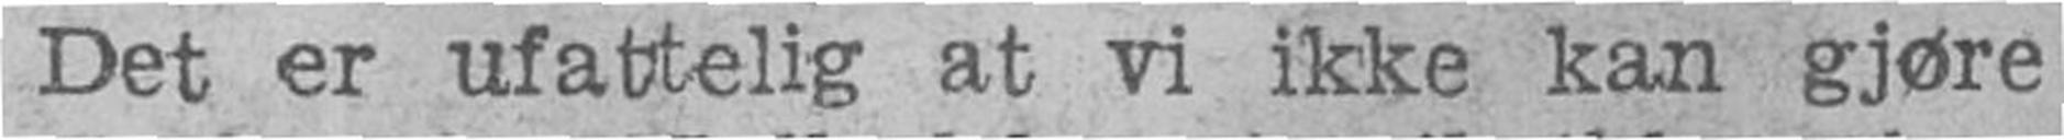Det er ufattelig at vi ikke kan gjøre
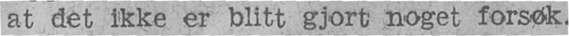at det ikke er blitt gjort noget forsøk.
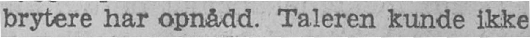brytere har opnådd. Taleren kunde ikke
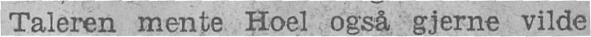Taleren mente Hoel også gjerne vilde
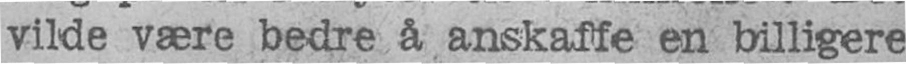vilde være bedre å anskaffe en billigere
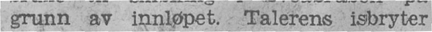grunn av innløpet. Talerens isbryter
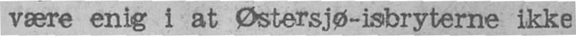være enig i at Østersjø-isbryterne ikke
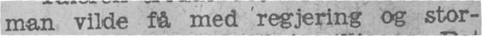man vilde få med regjering og stor-
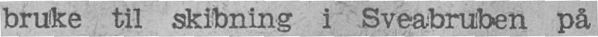bruke til skibning i Sveabruben på
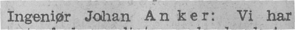Ingeniør Johan Anker: Vi har
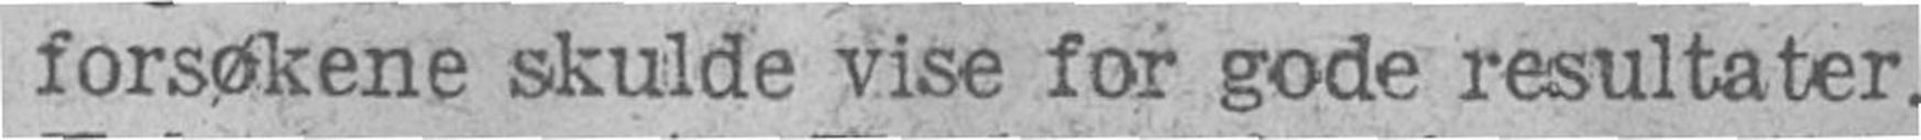forsøkene skulde vise for gode resultater.
